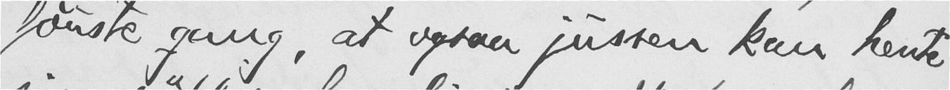første gang, at ogsaa jussen kan hente
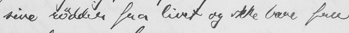sine rødder fra livet og ikke bare fra
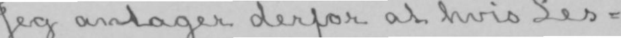Jeg antager derfor at hvis Les-
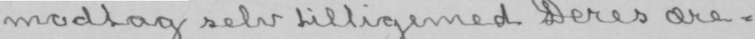modtag selv tilligemed Deres ære-
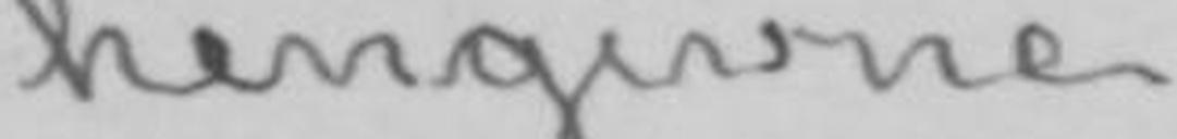hengivne
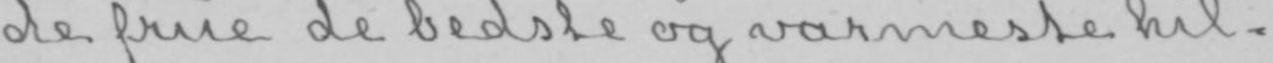de frue de bedste og varmeste hil-
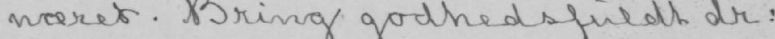næret. Bring godhedsfuldt dr:
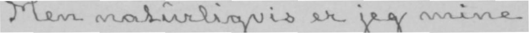Men naturligvis er jeg mine
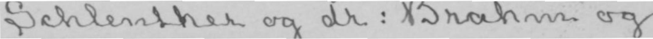Schlenther og dr: Brahm og
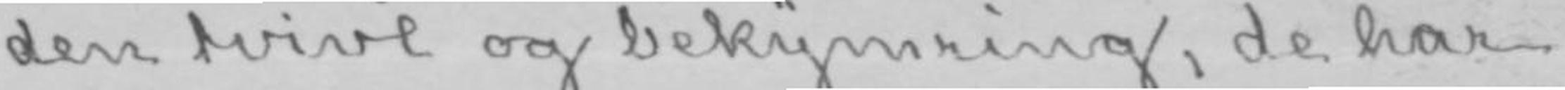den tvivl og bekymring, de har
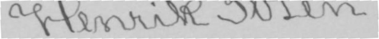Henrik Ibsen.
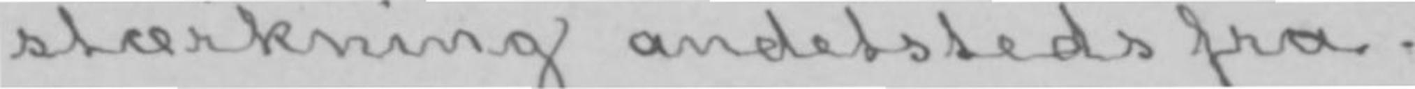stærkning andetsteds fra.
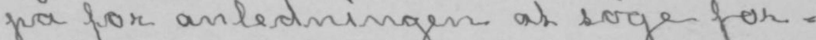på for anledningen at søge for-
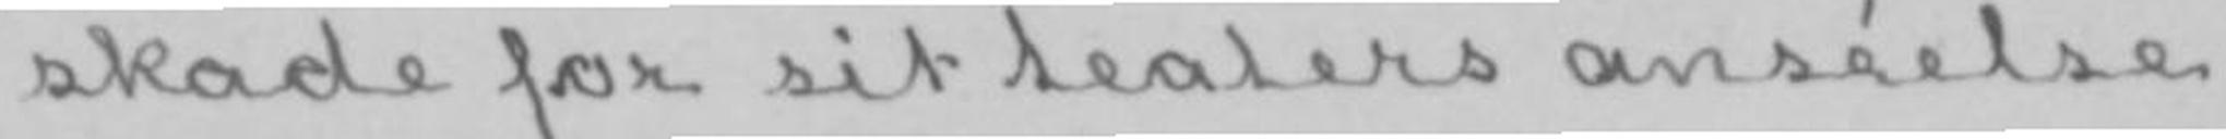skade for sit teaters anséelse.
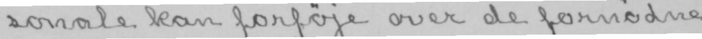sonale kan forføje over de fornødne
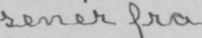sener fra
